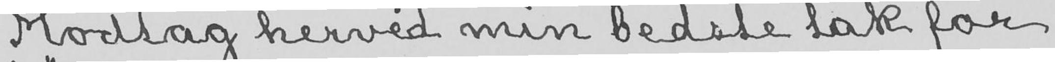Modtag herved min bedste tak for
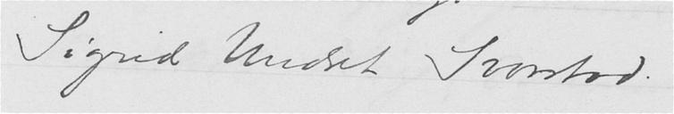Sigrid Undset Svarstad.
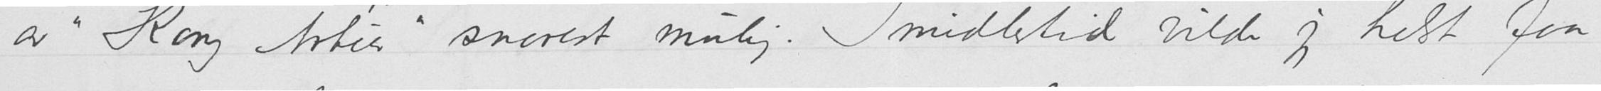av «Kong Artur» snarest mulig. Imidlertid vilde jeg helst faa
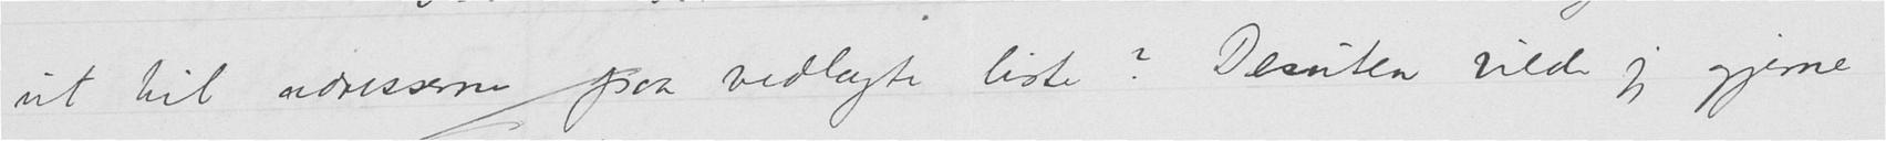ut til adresserne paa vedlagte liste? Desuten vilde jeg gjerne
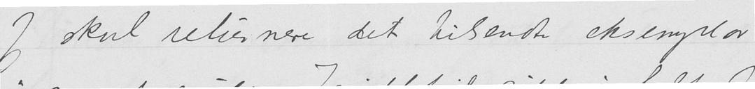Jeg skal returnere det tilsendte eksemplar
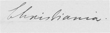Christiania.
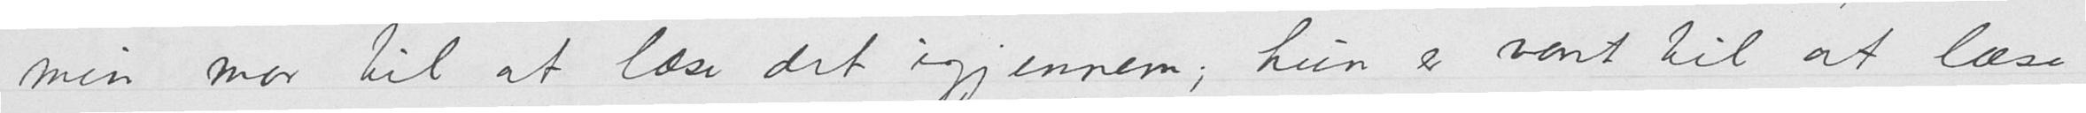min mor til at læse det igjennem; hun er vant til at læse
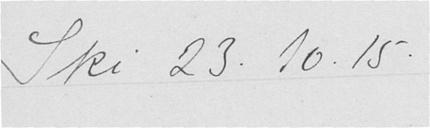Ski 23.10.15.
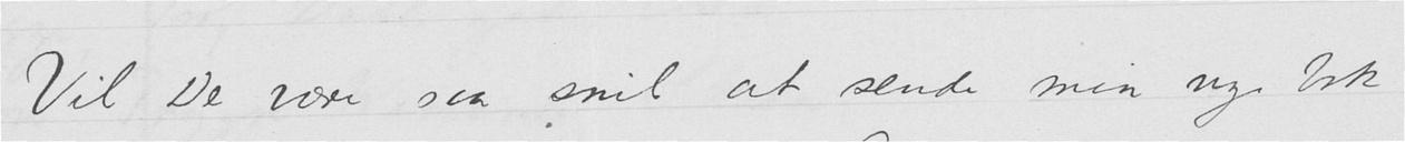Vil De være saa snil at sende min nye bok
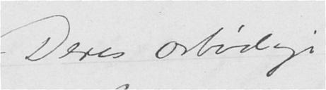Deres ærbødige
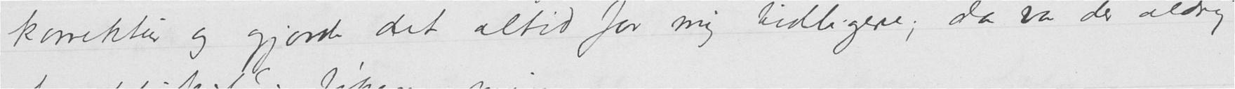korrektur og gjorde det altid for mig tidligere; da var der aldrig
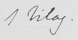1 bilag.
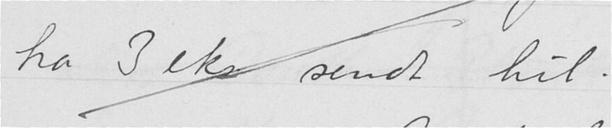ha 3 eks. sendt hit.
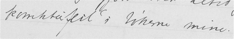korrekturfeil i bøkerne mine.
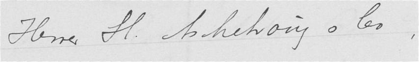Herrer H. Aschehoug o Co,
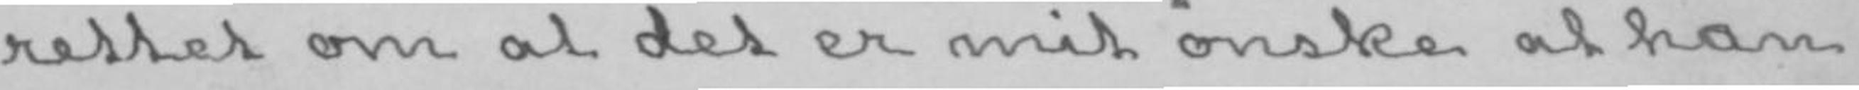rettet om at det er mit ønske at han
